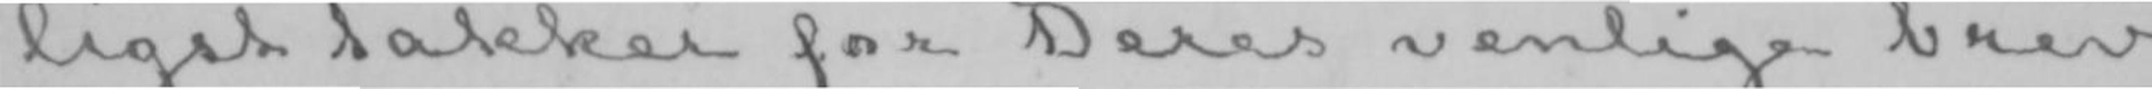ligst takker for Deres venlige brev
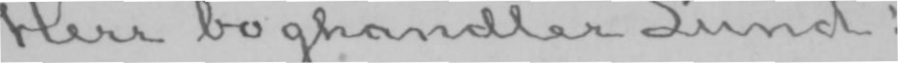Herr boghandler Lund!
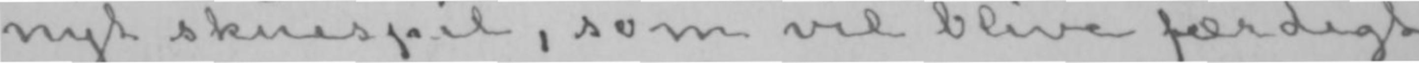nyt skuespil, som vil blive færdigt
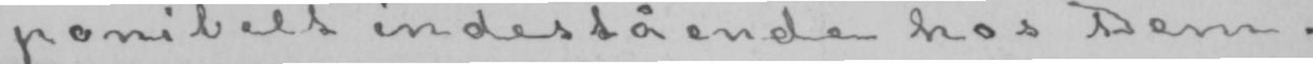ponibelt indestående hos Dem.
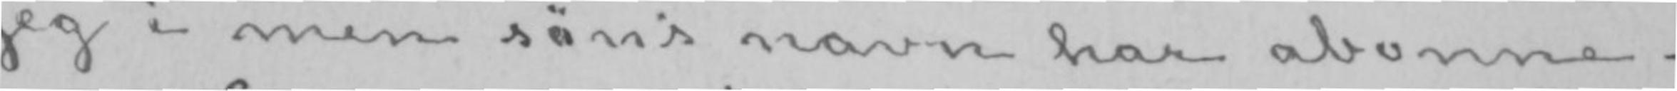jeg i min søns navn har abonne-
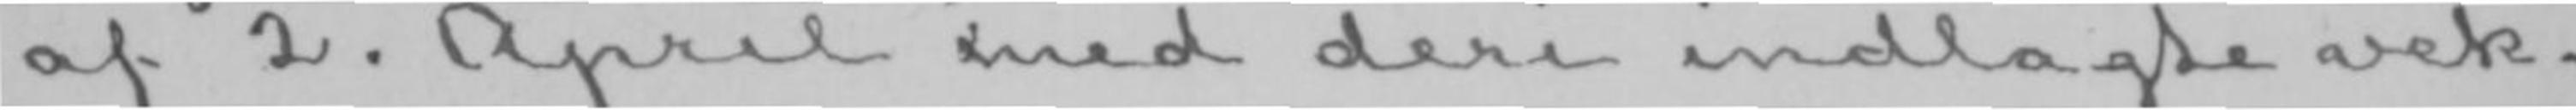af 2. April med deri indlagte vek-
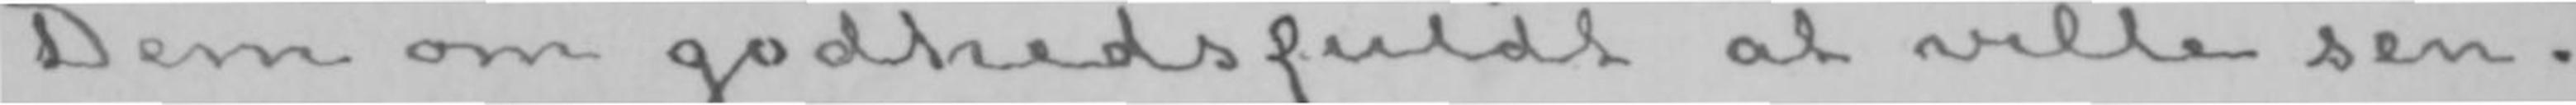Dem om godhedsfuldt at ville sen-
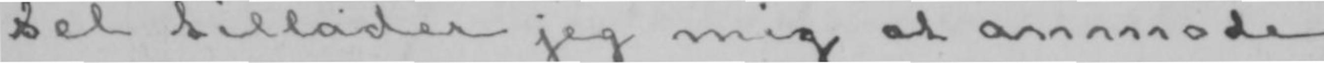sel tillader jeg mig at anmode
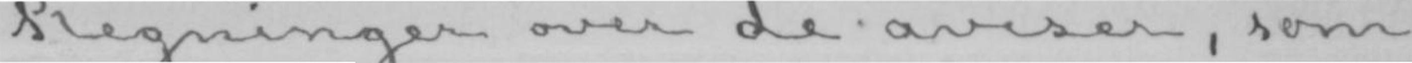Regningen over de aviser, som
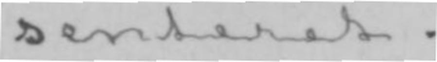senteret.
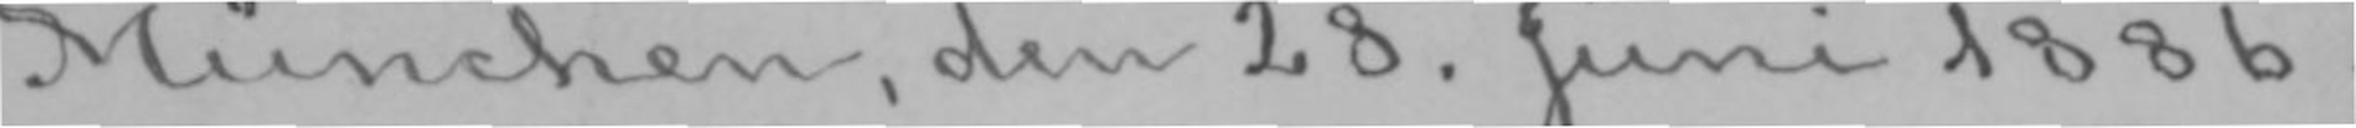München, den 28. Juni 1886.
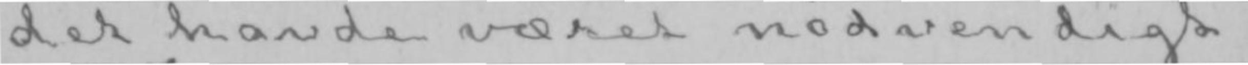det havde været nødvendigt.
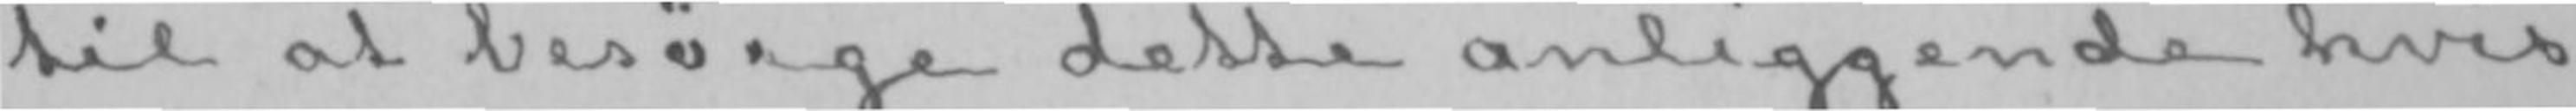til at besørge dette anliggende hvis
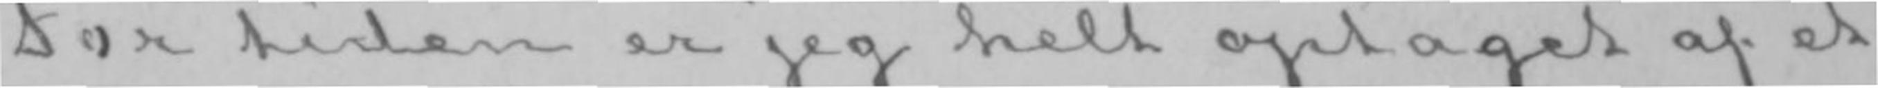For tiden er jeg helt optaget af et
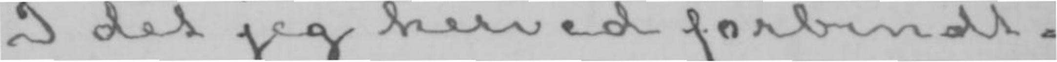I det jeg herved forbindt-
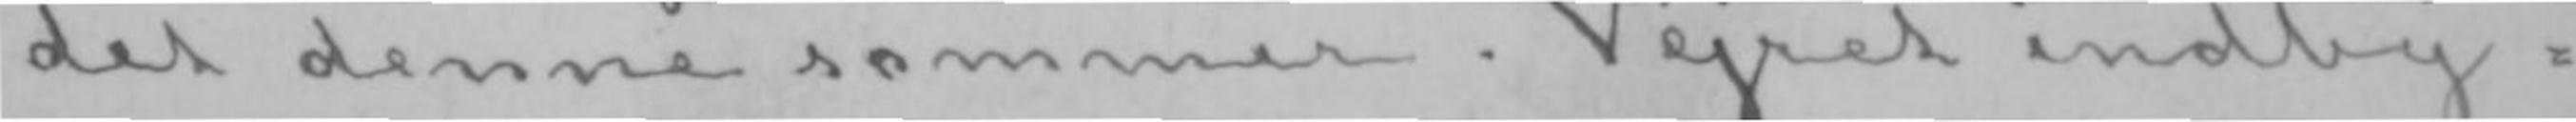det denne sommer. Vejret indby-
